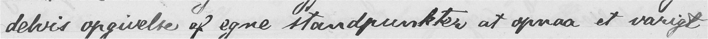delvis opgivelse af egne standpunkter at opnaa et varigt
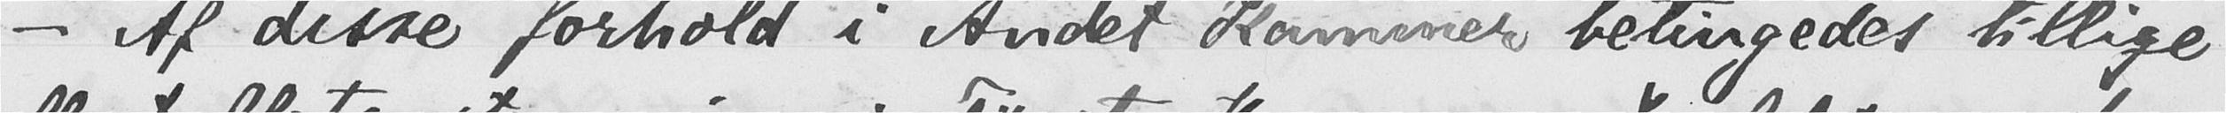- Af disse forhold i Andet Kammer betingedes tillige
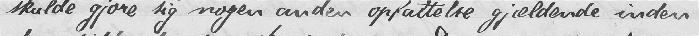skulde gjøre sig nogen anden opfattelse gjældende inden
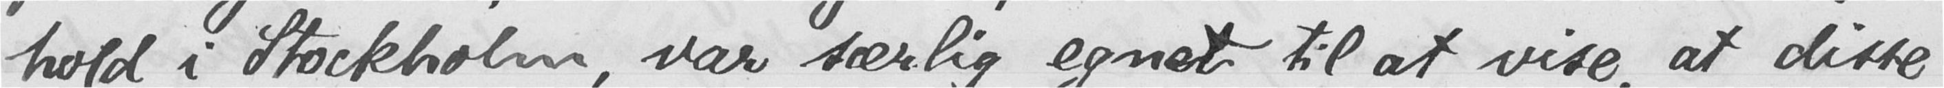hold i Stockholm, var særlig egnet til at vise, at disse
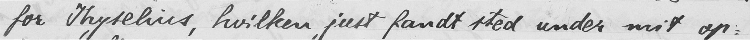for Thyselius, hvilken just fandt sted under mit op-
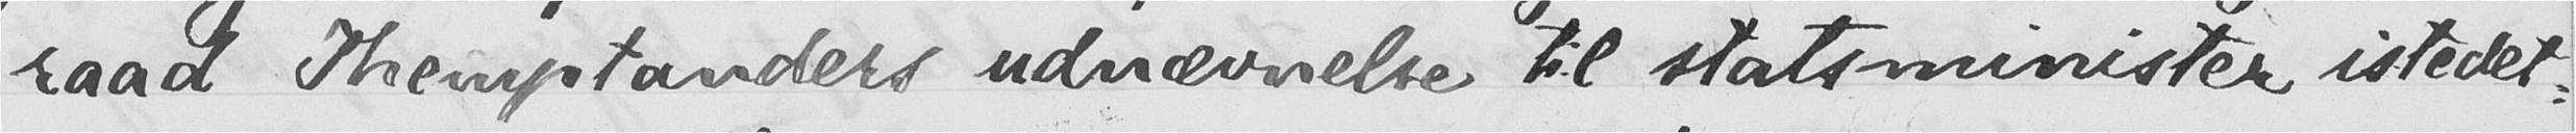raad Themptanders udnævnelse til statsminister istedet-
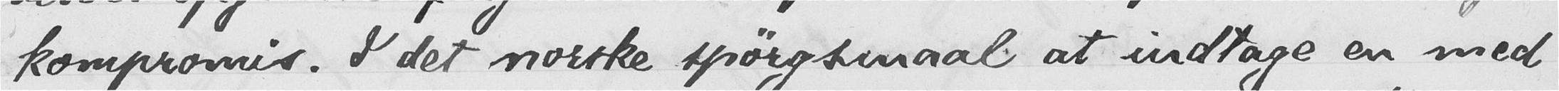kompromis. I det norske spørgsmaal at indtage en med
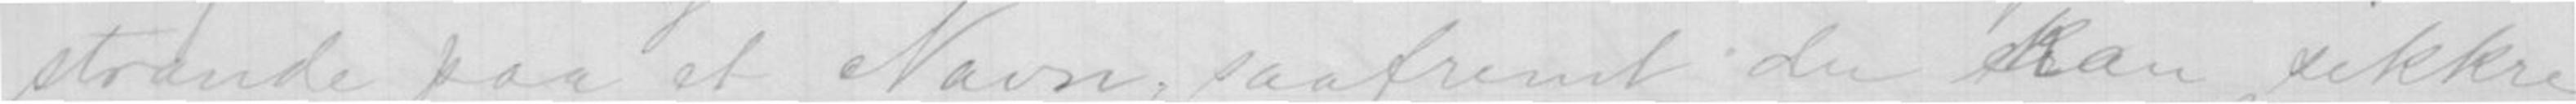strande pa et Navn, saafremt du kan sikkre
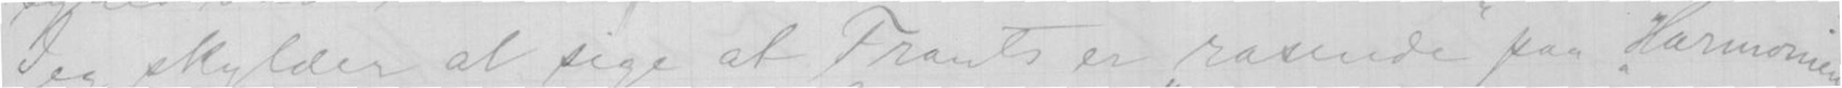Jeg skylder at sige at Frants er rasende paa Harmoniens
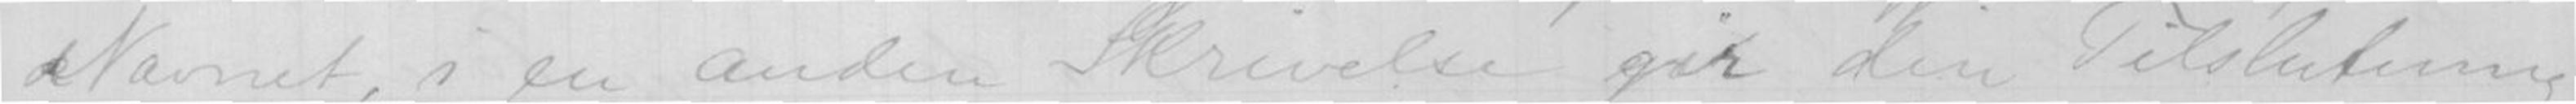Navnet, i en anden Skrivelse gir din Tilslutning
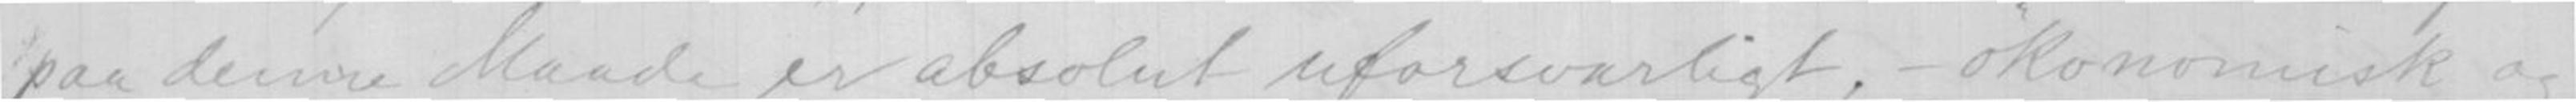paa denne Maade er absolut uforsvarligt, - økonomisk og
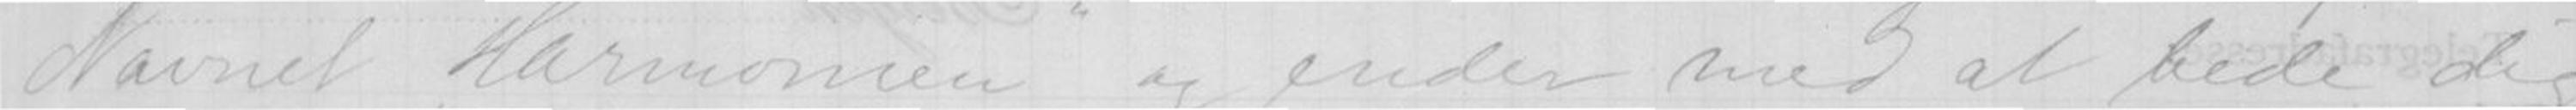Navnet Harmonien og ender med at bede dig
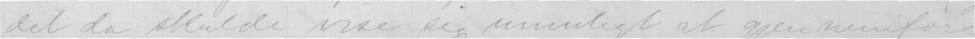det da skulde vise sig umuligt at gjennemføre
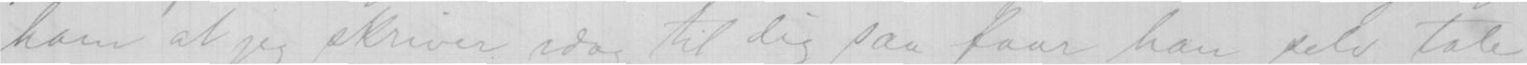ham at jeg skriver idag til dig saa faar han selv tale
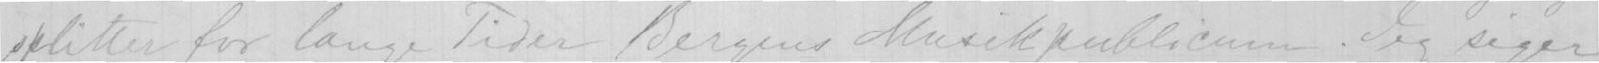splitter for lange Tider Bergens Musikpublicum. Jeg siger
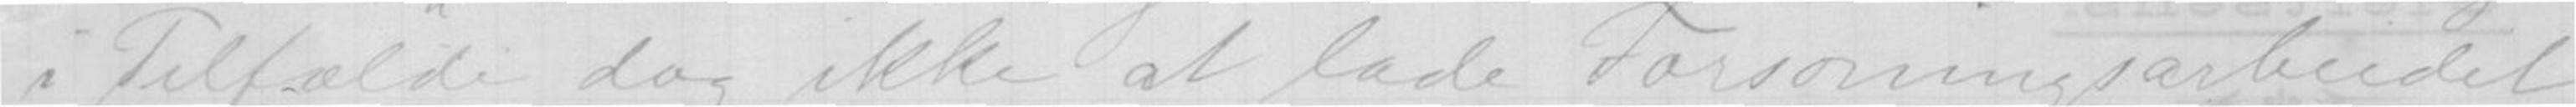i Tilfælde dog ikke at lade Forsoningsarbeidet
